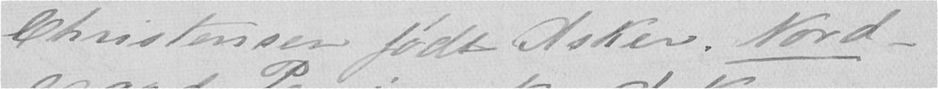Christensen født Asker Nord-
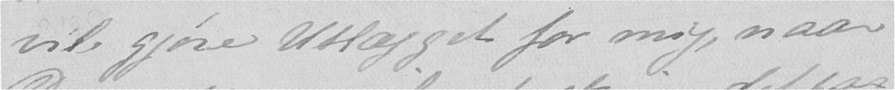vil gjøre Utlægget for mig, naar
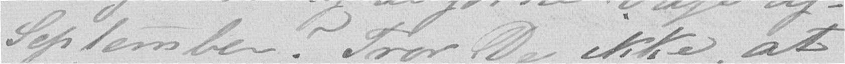September? Tror De ikke, at
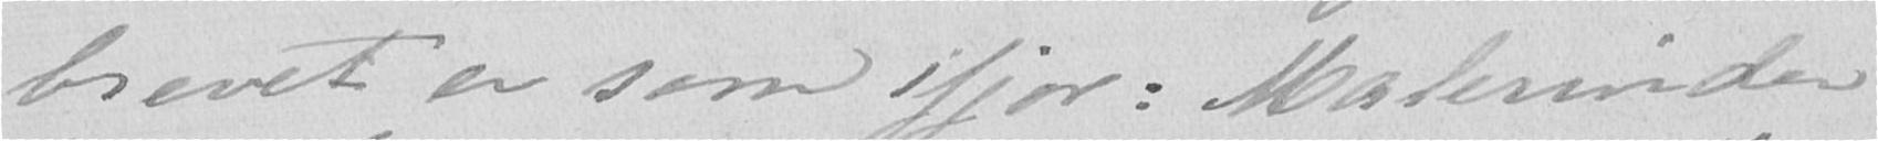brevet er som ifjor: Malerinden
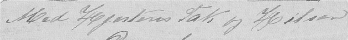Med Hjertens Tak og Hilsen
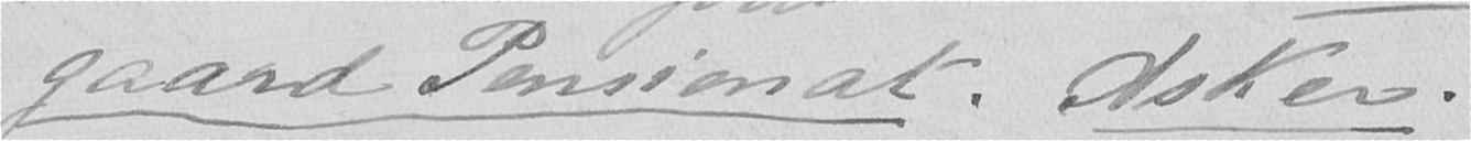gaard Pensionat. Asker.
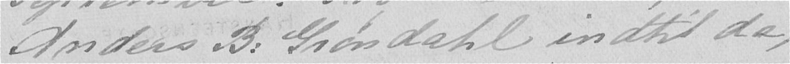Anders B. Grøndahl indtil da,
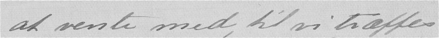at vente med, til vi træffes
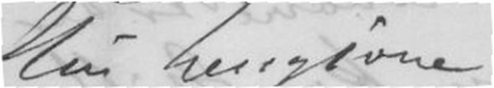Din hengivne
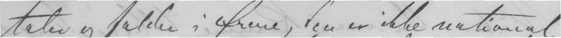taler og falder i Ørene, den er ikke national
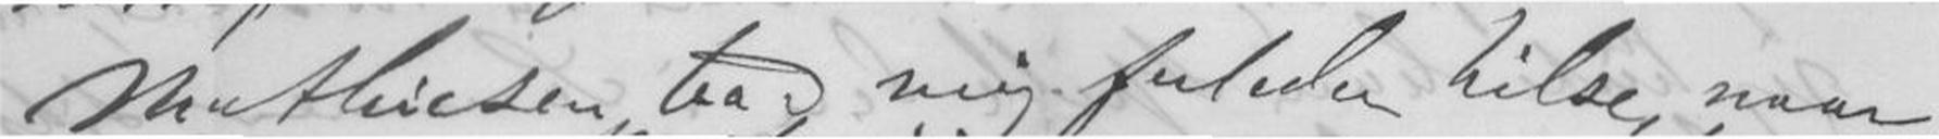Mathiesen bad mig forleden hilse, naar
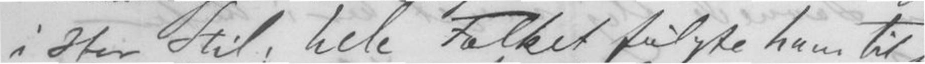i stor Stil; hele Folket fulgte ham til
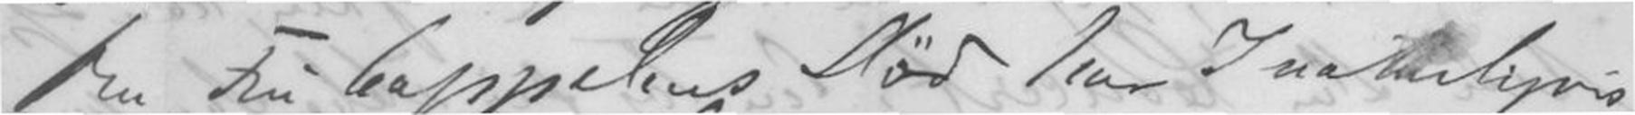Om Fru Cappelens Død har I naturligvis
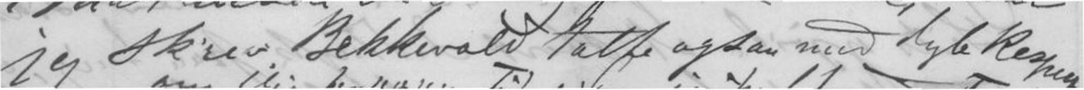jeg skrev Bekkevold talte ogsaa med dyb Respect
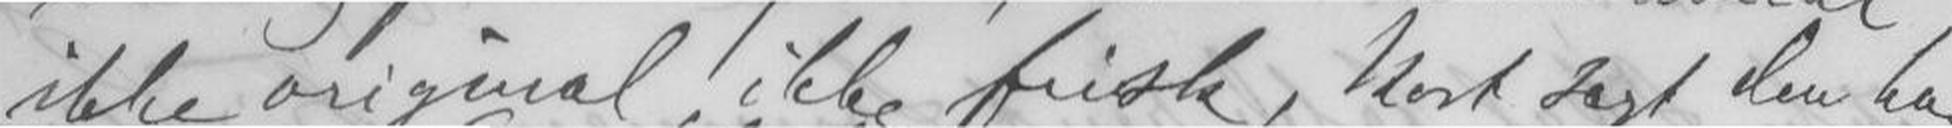ikke original, ikke frisk, kort sagt den har
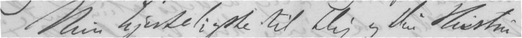Min hjerteligste til Dig og Din Hustru
